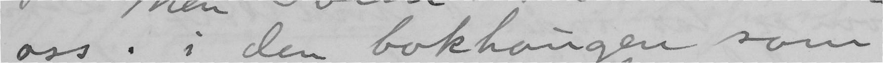oss i den bokhougen som
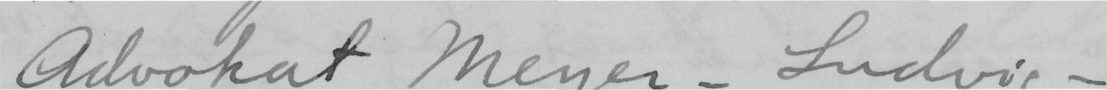Advokat Meyer-Ludvig –
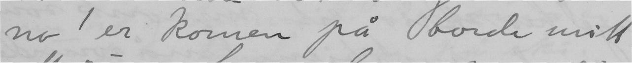no er komen på borde mitt
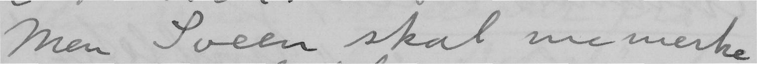Men Sveen skal me merke
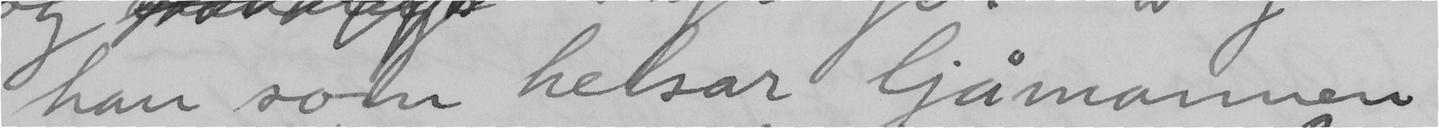han som helsar hjåmannen
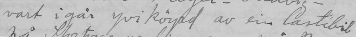vart i går yviköyrd av ein lastebil
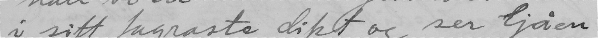i sitt fagraste dikt og ser hjåen
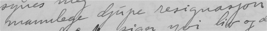mannlege djupe resignasjon
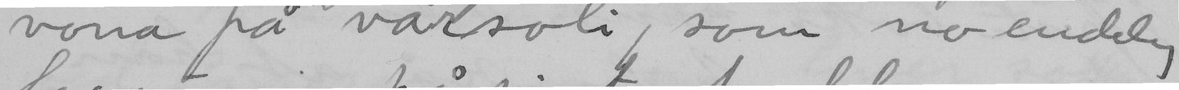vona på vårsoli, som no endeleg
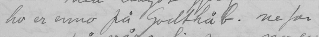ho er enno på Godthåb. Me for
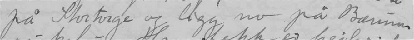på Stortorge og ligg no på Bærum
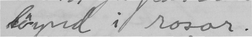göymd i rosor.
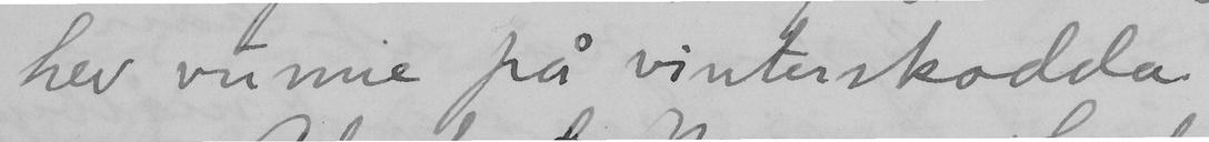hev vunne på vinterskodda.
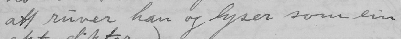att ruver han og lyser som ein
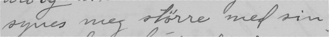synes meg større med sin
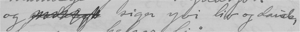og  siger yvi liv og daud,
